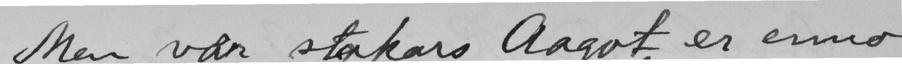Men vår stakars Aagot er enno
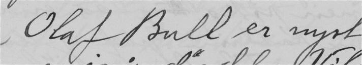Olaf Bull er nyst
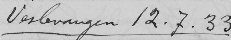Veslevangen 12.7.33
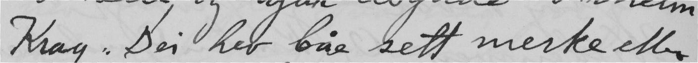Krag. Dei hev båe sett merke etter
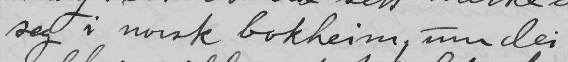set i norsk bokheim um dei
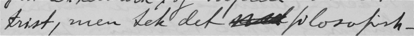trist, men tek det  filosofisk –
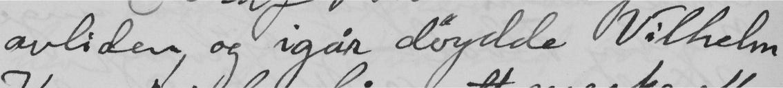avliden, og igår döydde Vilhelm
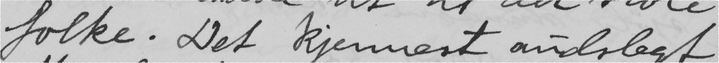folke. Det kjennest audslegt
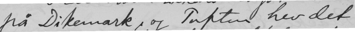på Dikemark, og Tuften hev det
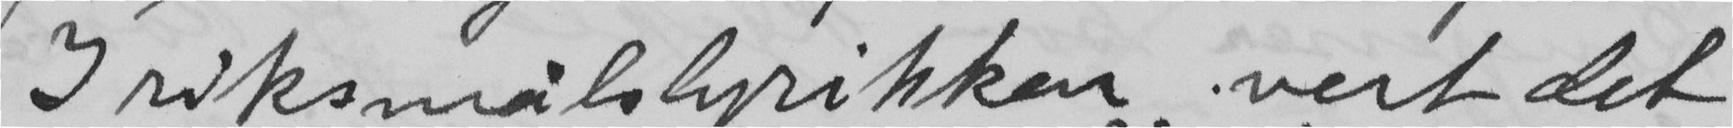I riksmålslyrikken vert det
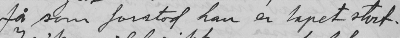få som forstod han er tapet stort.
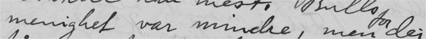menighet var mindre, men for dei
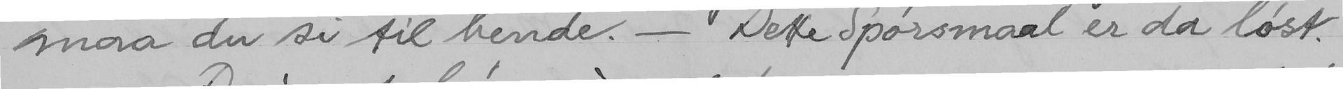maa du si til hende. - Dette Spørsmaal er da løst.
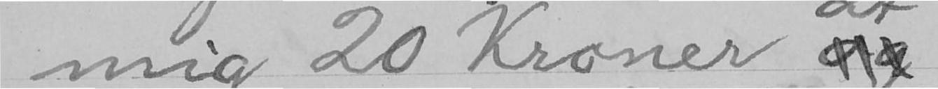mig 20 Kroner og
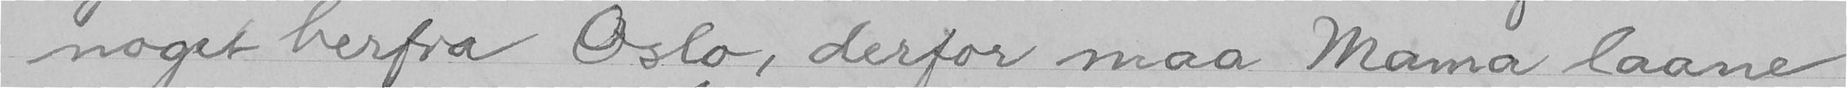noget herfra Oslo, derfor maa Mama laane
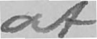at
